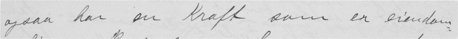ogsaa har en Kraft som er eiendom-
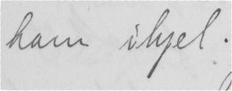ham ihjel.
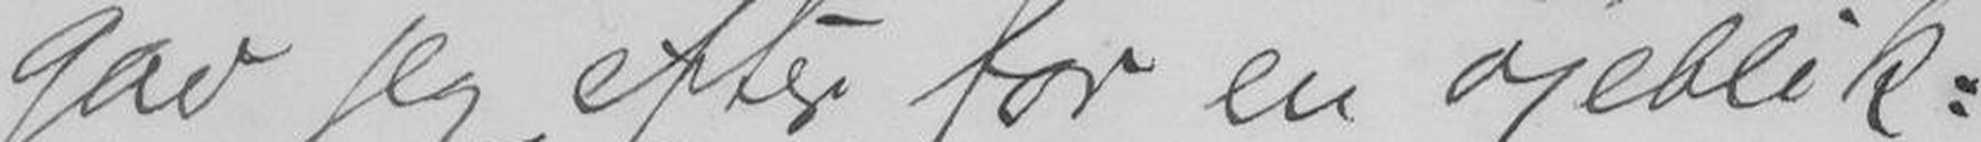gav jeg efter for en øjeblik-
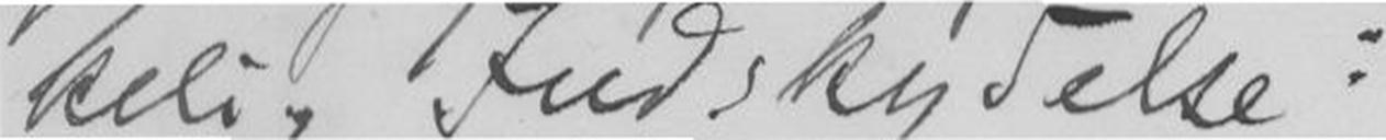kelig Indskydelse:
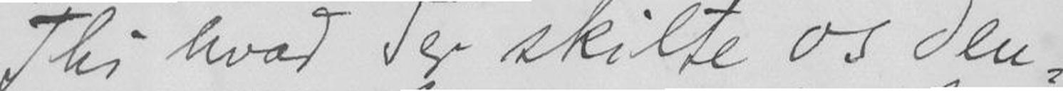Thi hvad der skilte os den-
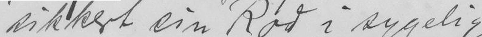sikkert sin Rod i sygelig
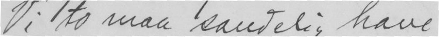Vi to maa sandelig have
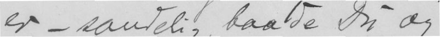er - sandelig, baade Du og
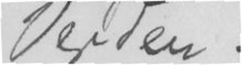Verden!
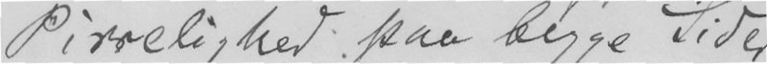Pirrelighed paa begge Sider
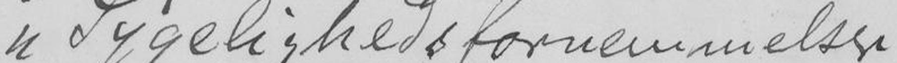Sygelighedsfornemmelser
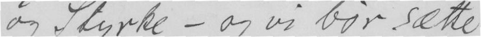og Styrke - og vi bør sætte
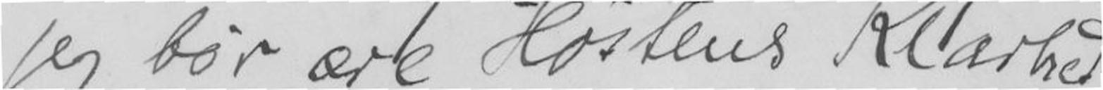jeg bør ære Høstend Klarhed
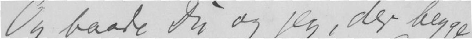Og baade Du og jeg, der begge
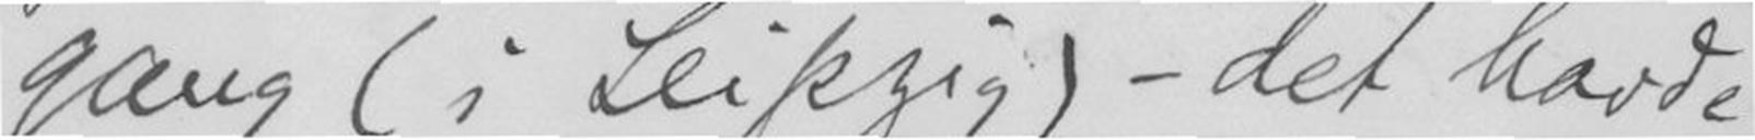gang (i Leipzig) - det havde
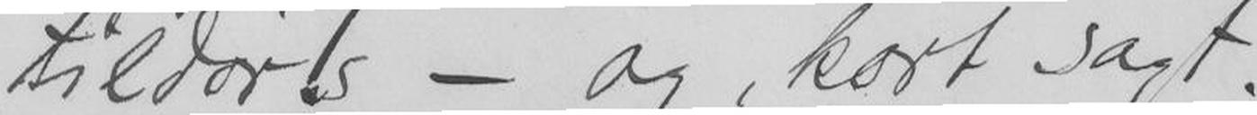tildørs - og, kort sagt:
